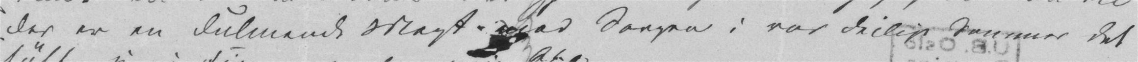der er en dulmende Magt – mod Sorgen i vor deilige Sommer det
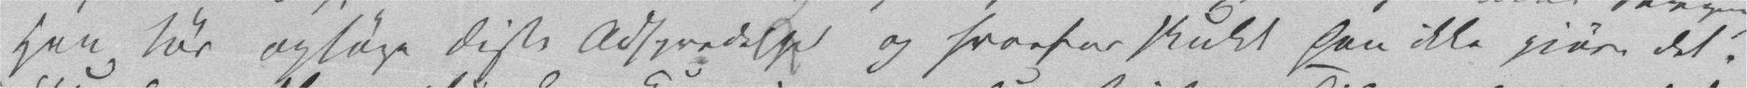Han tør opsøge disse Adspredelser og hvorfor skulde han ikke gjøre det?
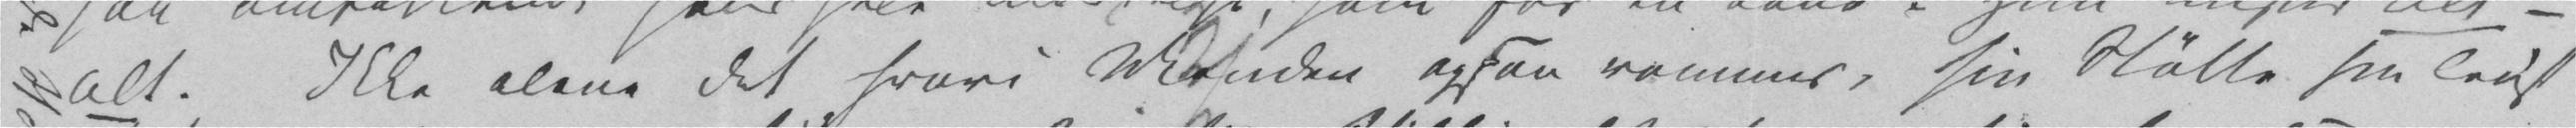Alt. Ikke alene det hvori Manden ogsaa rammes, sin Støtte sin Trøst
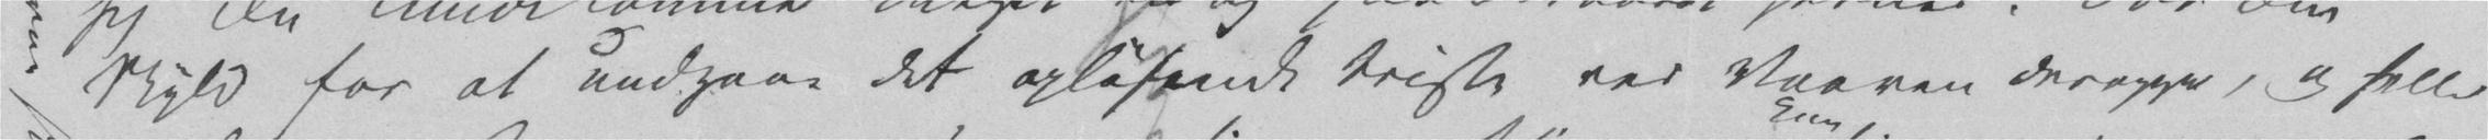Skyld for at undgaae det opløsende triste ved Vaaren deroppe, og heller
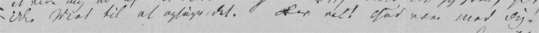ikke Mod til at opsøge det. Lev vel! Gud være med Dig!
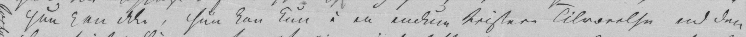Hun kan ikke, hun kan kun i en endnu tristere Tilværelse end den
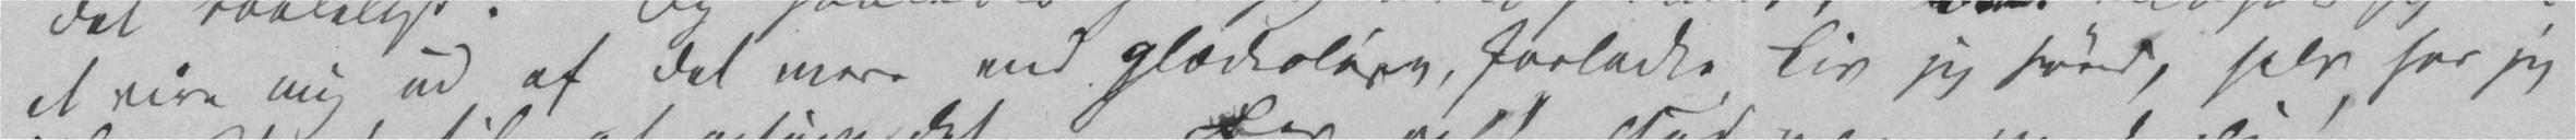at rive mig ud af det mere end glædesløse, forladte Liv jeg fører, selv har jeg
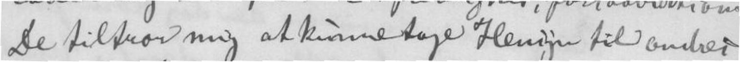De tiltror mig at kunne tage Hensyn til andres
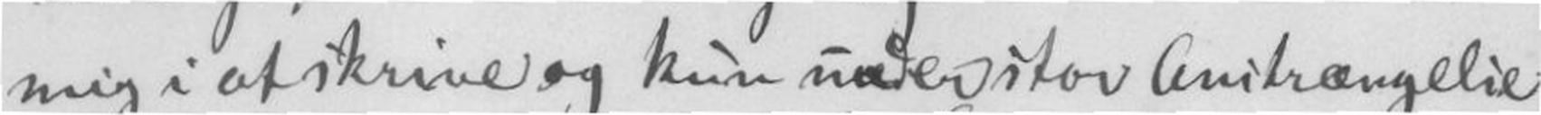mig i at skrive og kun under stor Anstrængelse
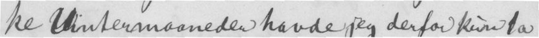ke Vintermaaneder havde jeg derfor kun 1 a
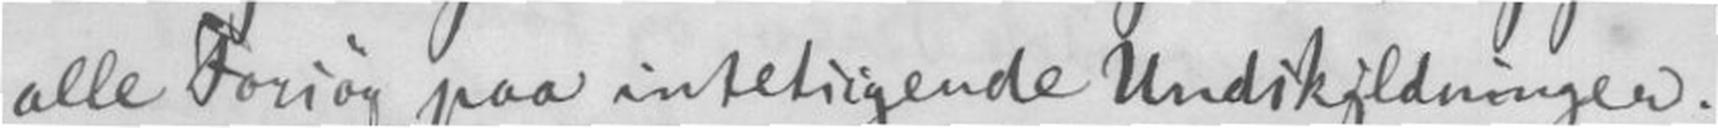alle Forsøg paa intetsigende Undskyldninger.
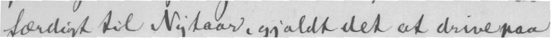færdigt til Nytaar, gjaldt det at drive paa
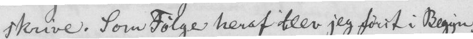skrive. Som Følge heraf blev jeg først i Begyn-
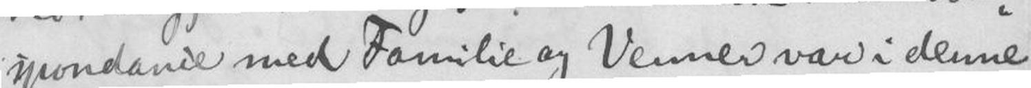spondanse med Familie og Venner var i denne
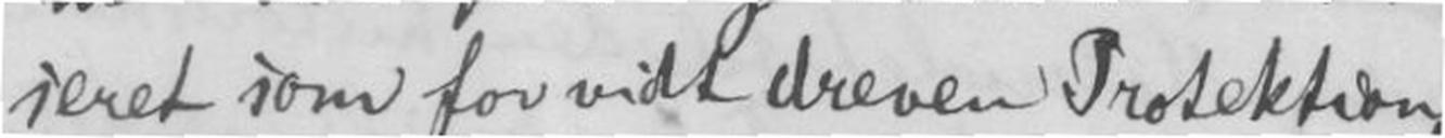seret som for vidt dreven Protektion
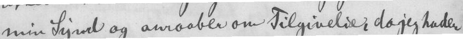min Synd og anraaber om Tilgivelse, da jeg hader
